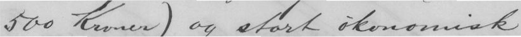500 Kroner) og stort økonomisk
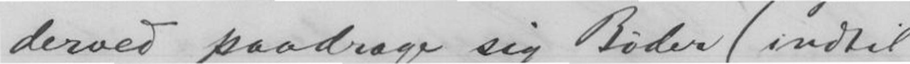derved paadrage sig Bøder (indtil
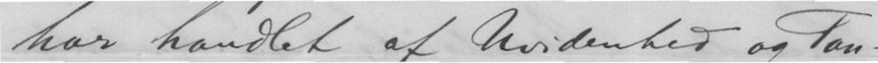har handlet af Uvidenhed og Tan-
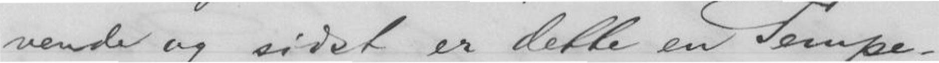vende og sidst er dette en Tempe-
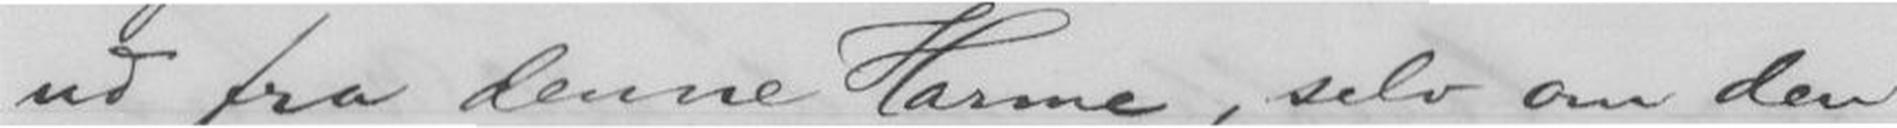ud fra denne Harme, selv om den
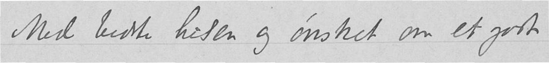Med bedste hilsen og ønsket om et godt
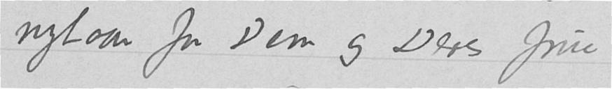nytaar for Dem og Deres frue
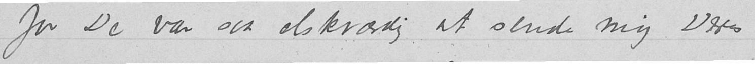for De var saa elskværdig at sende mig Deres
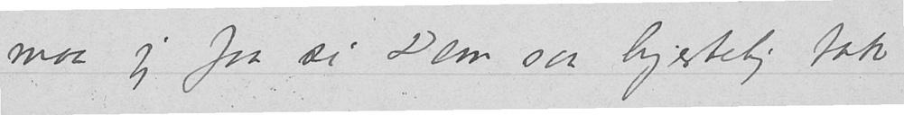maa jeg faa si Dem saa hjertelig tak
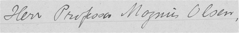Herr Professor Magnus Olsen,
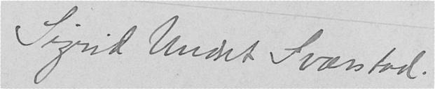Sigrid Undset Svarstad.
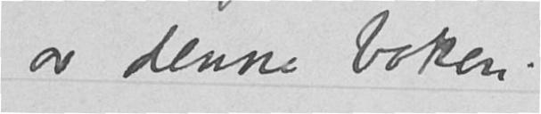av denne boken.
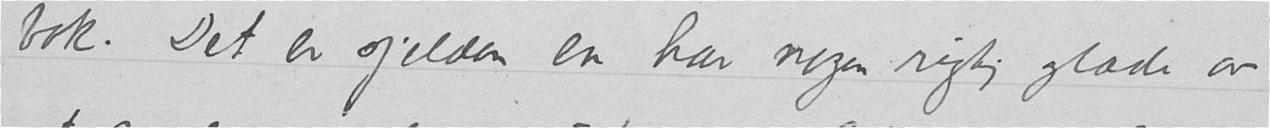bok. Det er sjelden en har nogen rigtig glæde av
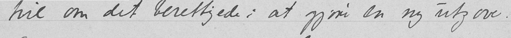tvil om det berettigede i at gjøre en ny utgave.
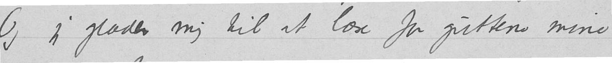Og jeg glæder mig til at læse for guttene mine
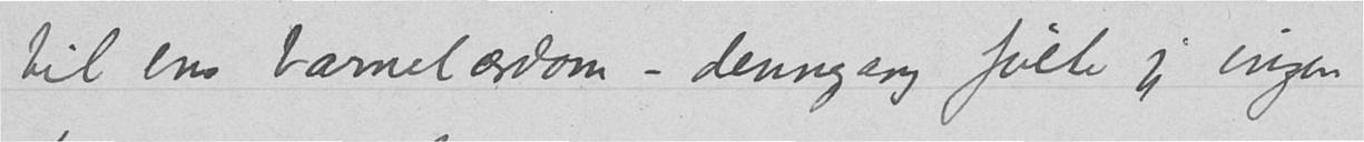til ens barnelærdom – dennegang følte jeg ingen
